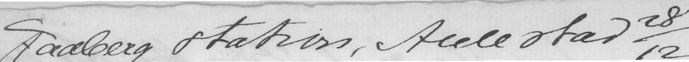Faaberg station, Aulestad 28/12
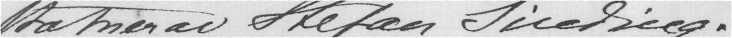statuer av Stefan Sinding.
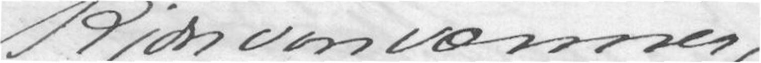Kjære vore vænner,
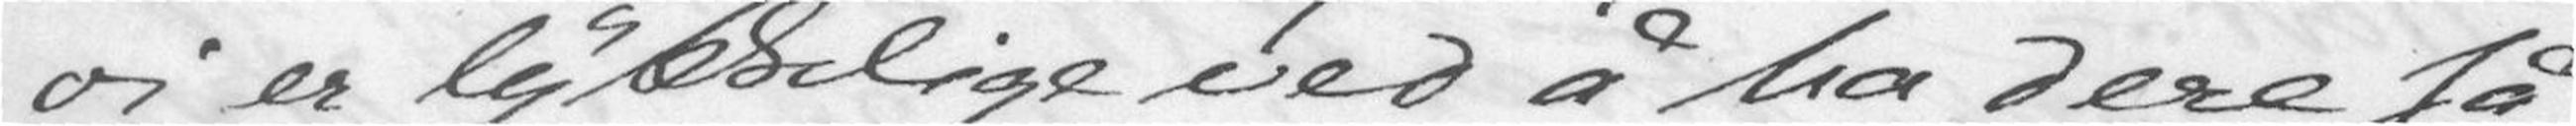vi er lykkelige ved å ha dere så
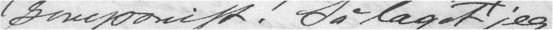komponist! Så laget jeg
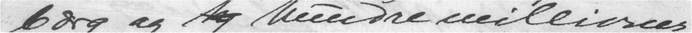bærg og hundrede
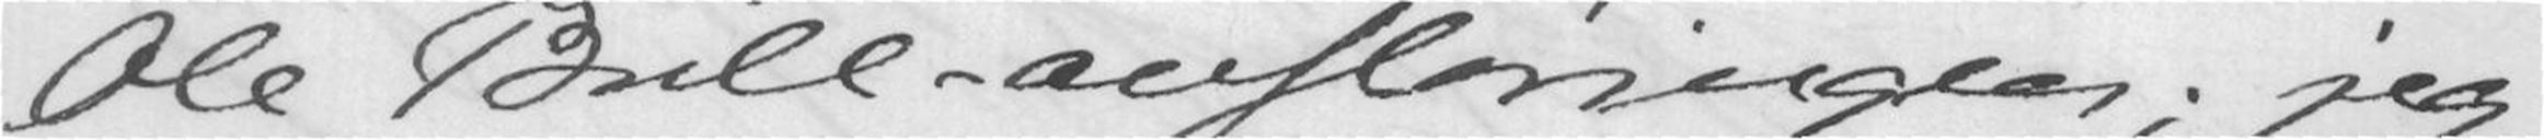Ole Bull-avsløringen; jeg
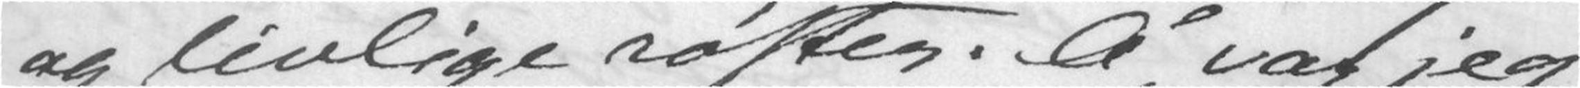og livlige røster. Å, var jeg
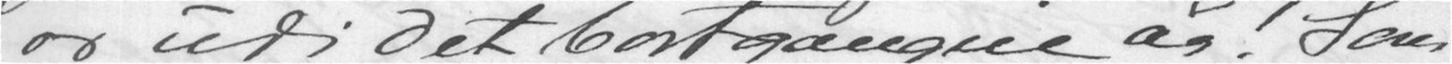udi det bortgangne år! Som
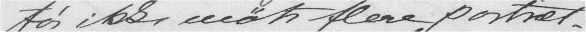tør ikke møte flere portræt-
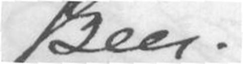Jeg kan hilse fra det
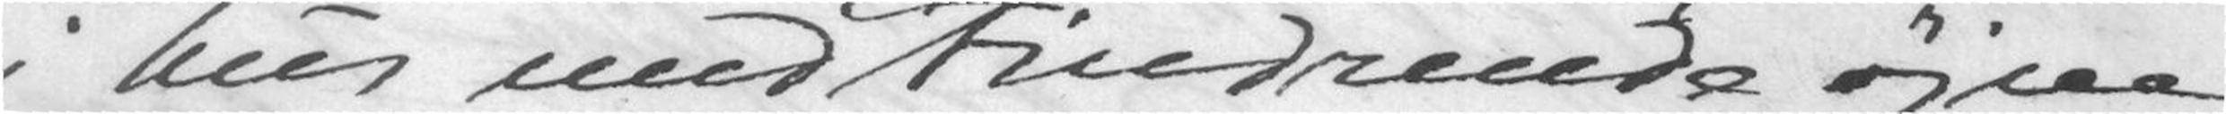i hus med tindrende øjne
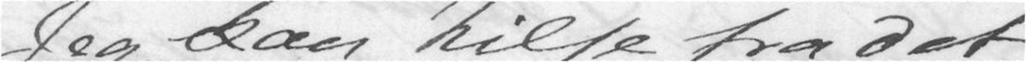sken.
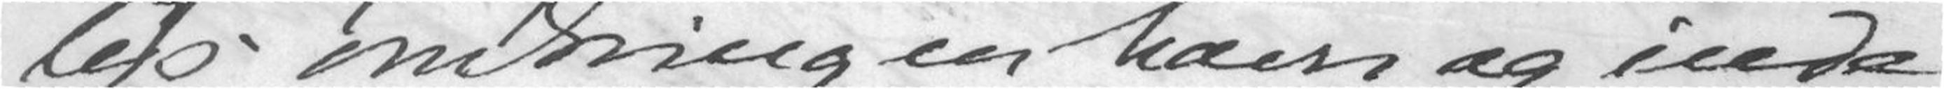millioner lys omkring en havn og inde
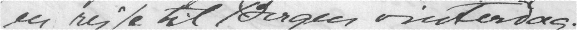en rejse til Bergen vinterdag.
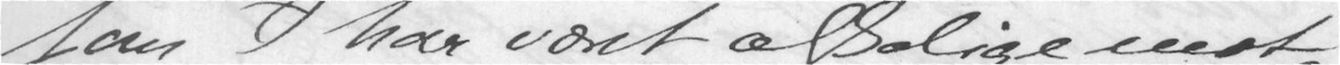som I har været ælskælige mot os
